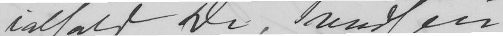ialfald De, Svendsen
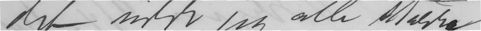det vilde jeg alle større
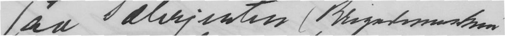saa Sæterjenten (Brigademusiken)
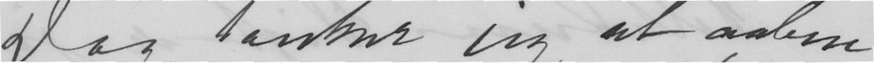Dog tænker jeg at aabne
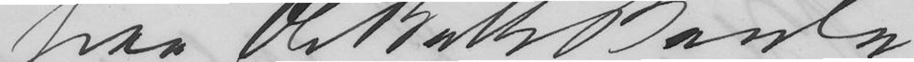paa Ole Bulls Bauta;
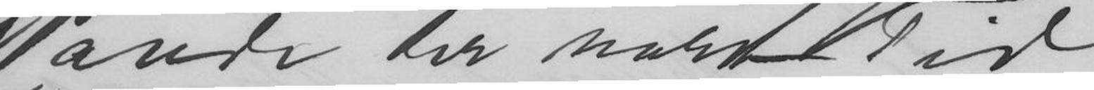Havde der været Tid
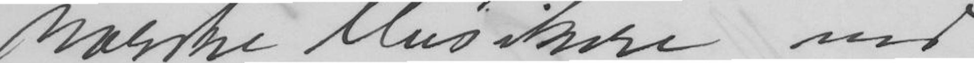norske Musikere ved
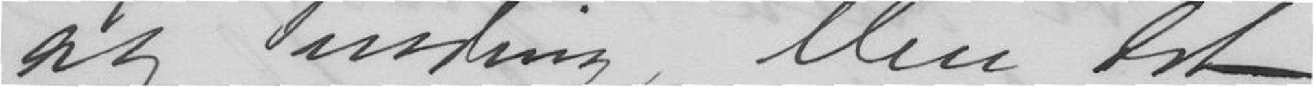og Sinding. Men det
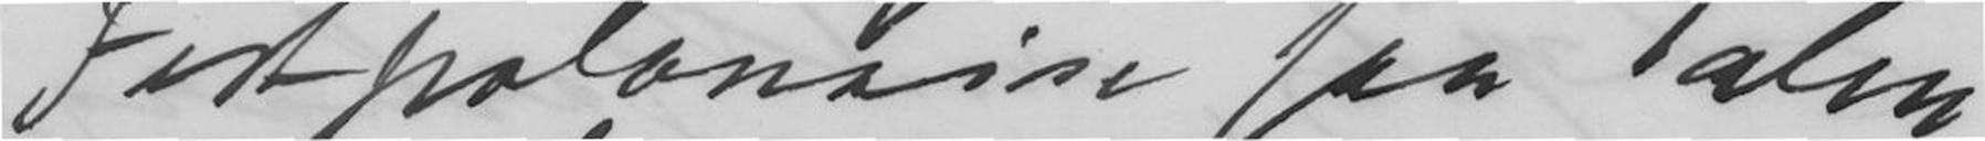Festpolonaise saa Talen
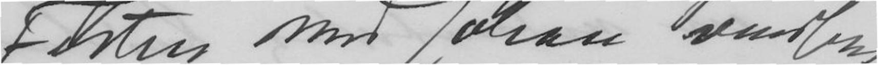Festen med Johan Svendsen
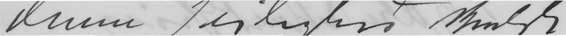denne Leilighed skulde
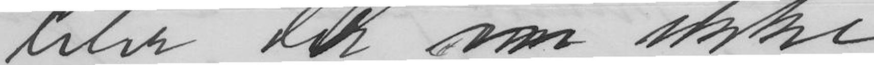blir der nu ikke
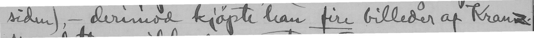siden), - derimod kjøpte han fire billeder af Kranz.
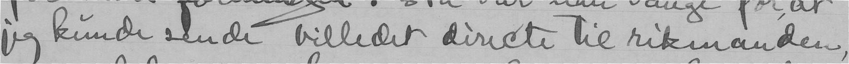jeg kunde sende billedet directe til rikmanden,
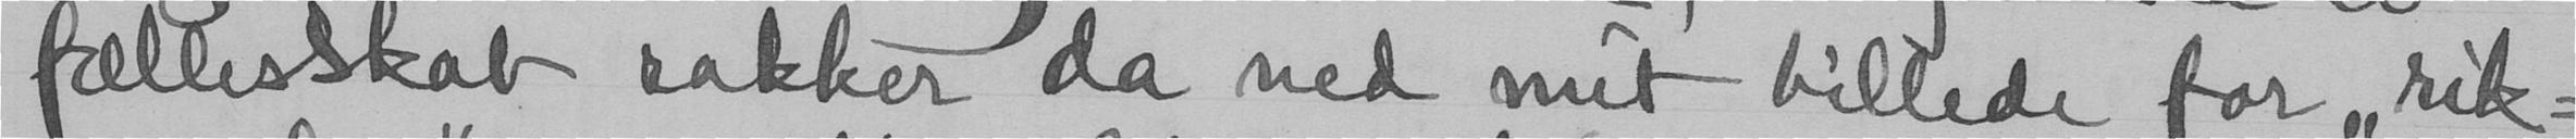fællesskab rakker da ned mit billede for "rik-
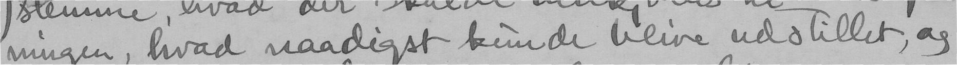eningen, hvad naadigst kunde blive udstillet, og
